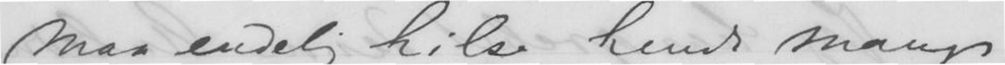maa endelig hilse hende mange
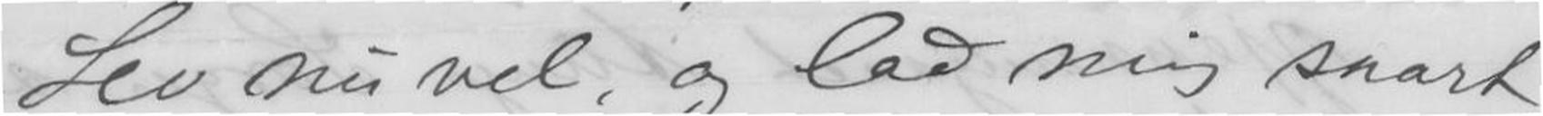Lev nu vel, og lad mig snart
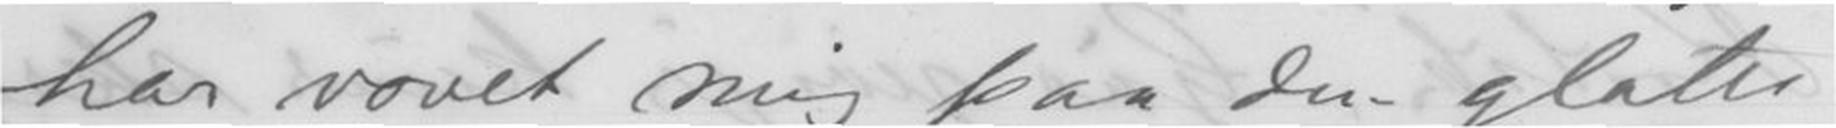har vovet mig paa den glatte
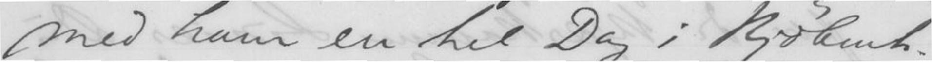med ham en hel Dag i Kjøbenh.
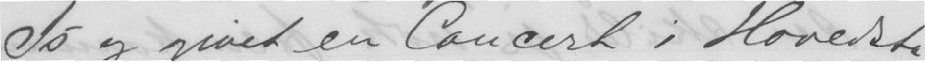Is og givet en Concert i Hovedsta-
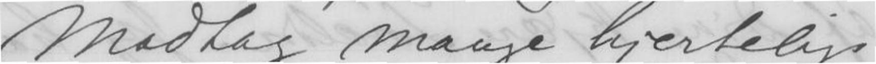Modtag mange hjertelige
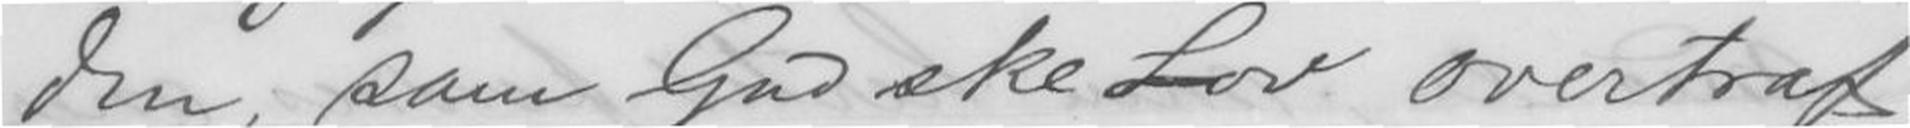den, som Gudske Lov overtraf
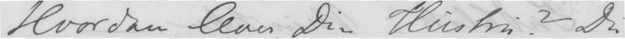Hvordan lever Din Hustru? Du
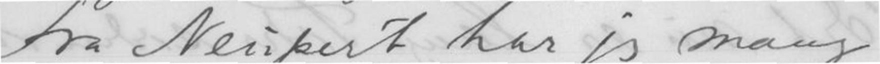Fra Neupert har jeg
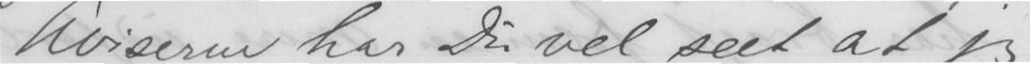Aviserne har Du vel seet at jeg
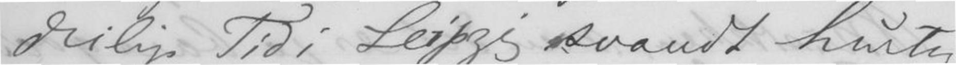deilige Tid i Leipzig svandt hurtig
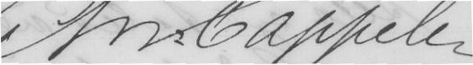Chr. Cappelen
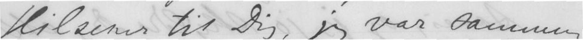mang Hilsener til Dig, jeg var sammen
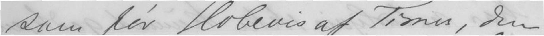som før Hobevis af Timer, den
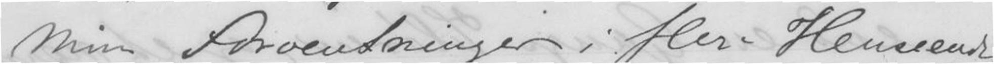min Forventninger i fler Henseende.
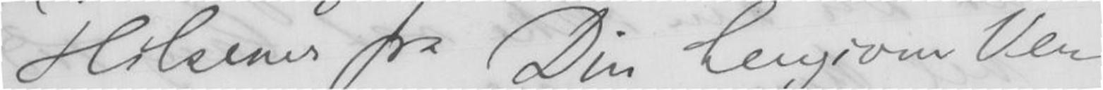Hilsener fra Din hengivne Ven
